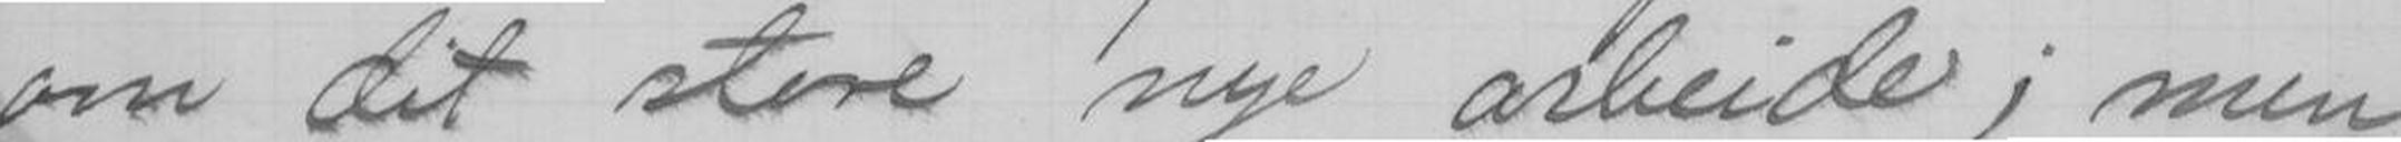om det store nye arbeide; men
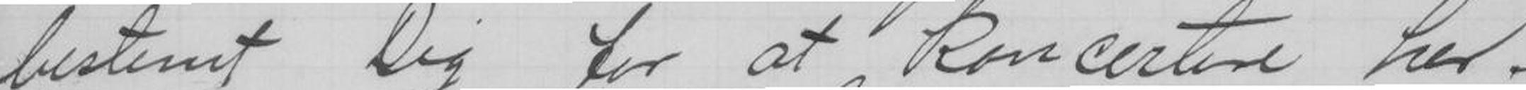bestemt Dig for at koncertere her.
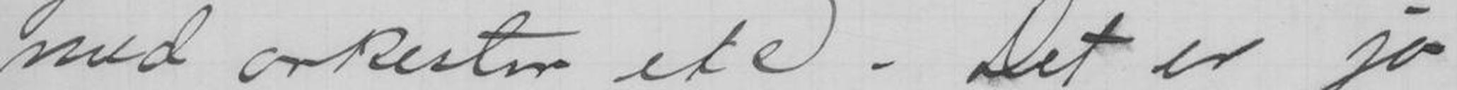med orkaster etc. Det er jo
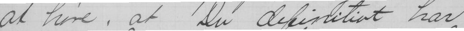at høre, at Du definitivt har
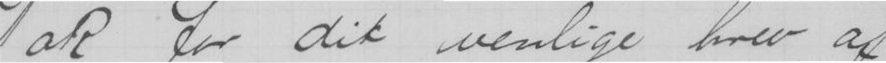Tak for dit venlige brev af
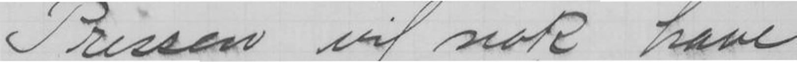Pressen vil nok have
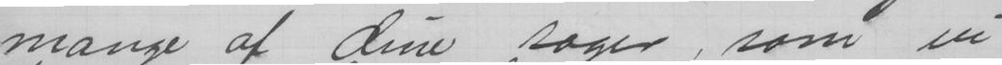mange af dine sager, som vi
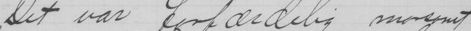Det var forfærdelig morsomt
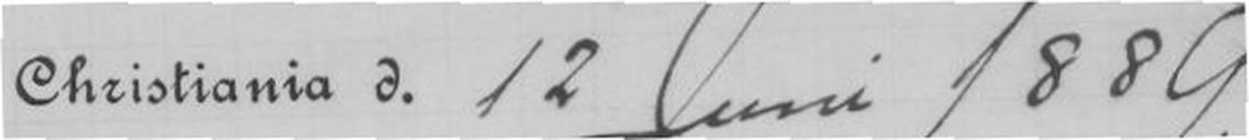Christiania d. 12 Juni 1889.
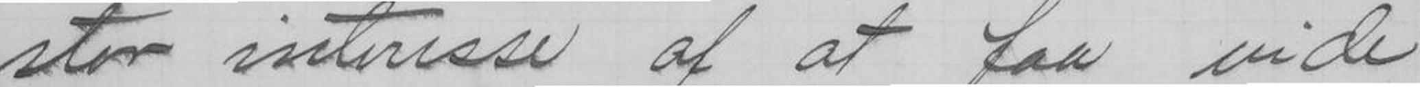stor interesse af at faa vide
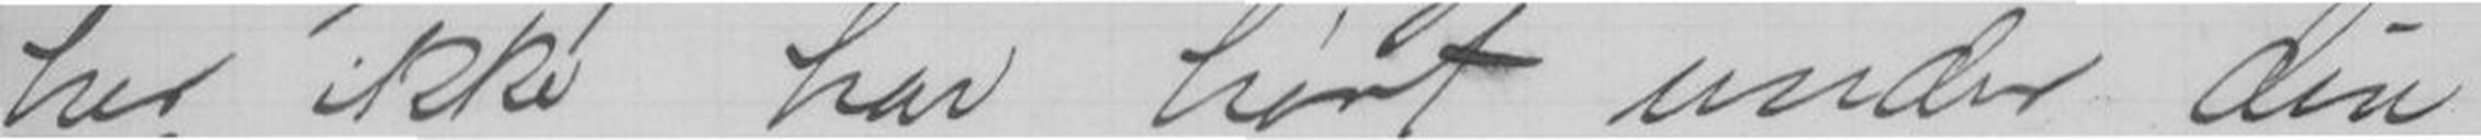her ikke har hørt under din
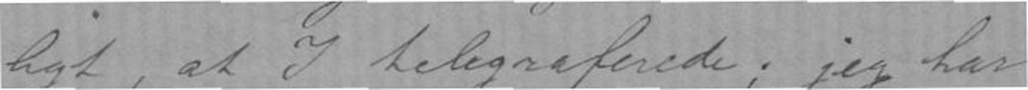ligt, at I telegraferede; jeg har
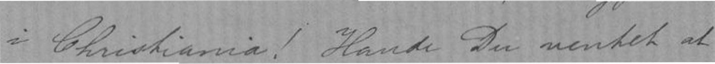i Christiania! Havde Du ventet at
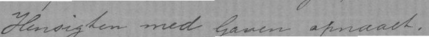Hensigten med Gaven opnaaet.
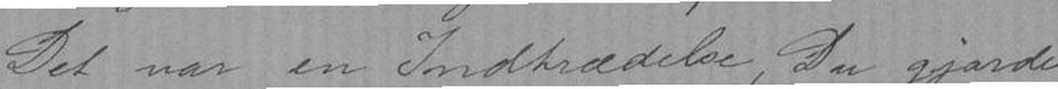Det var en Indtrædelse, Du gjorde
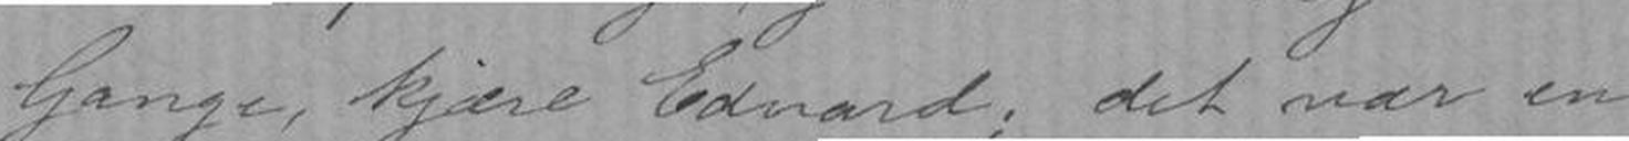Gange, kjære Edvard; det var en
